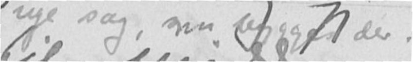nye sag, som bygges der.
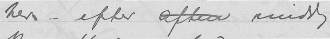her. - efter aften middag
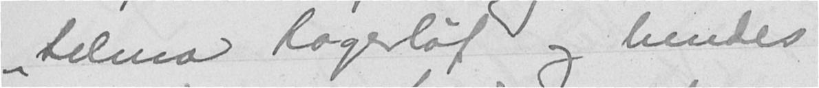"Selma Lagerløf og hendes
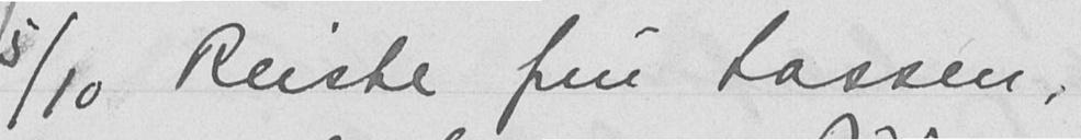5/10 Reiste fru Lassen,
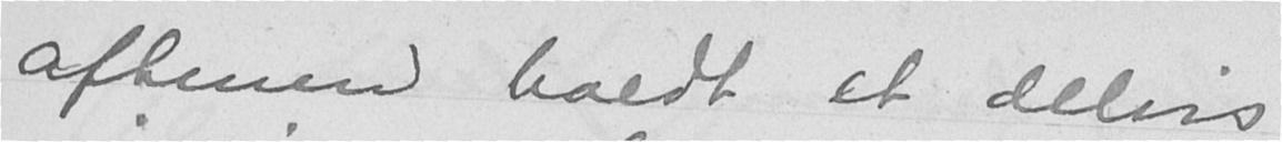aftenen holdt et delvis
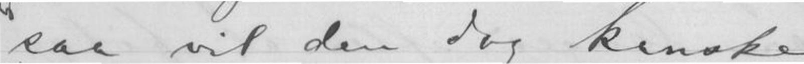saa vil den dog kanske
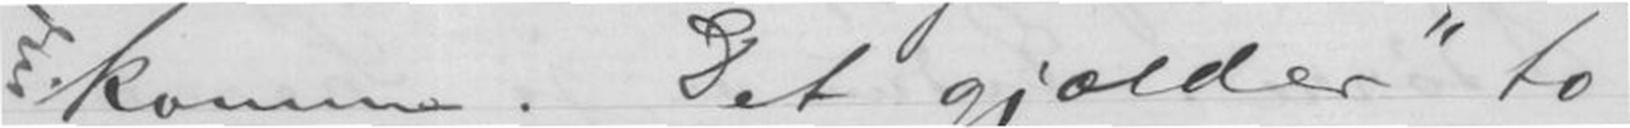komme. Der gjælder "to
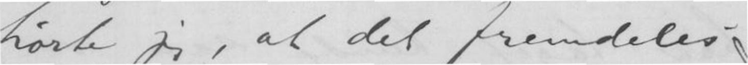hørte jeg, at det fremdeles
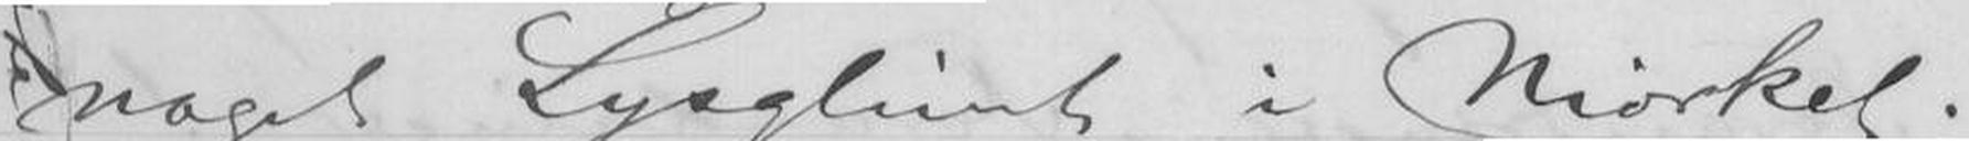noget Lystglimt i Mørket.
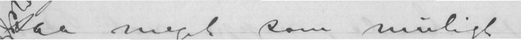saa meget som muligt og
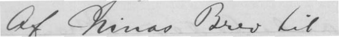Af Ninas Brev til
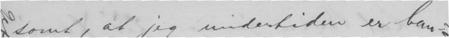somt, at jeg undertiden er ban-
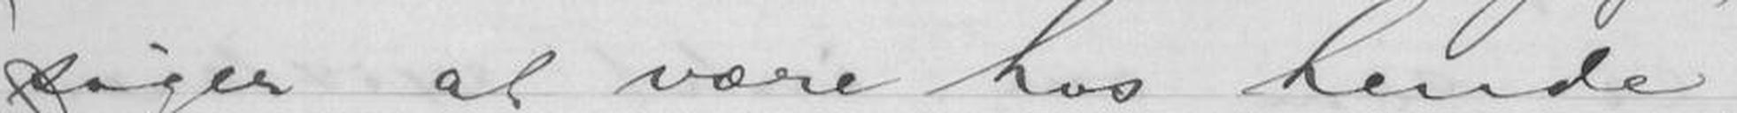søger at være hos hende
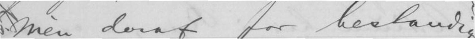Mèn deraf for bestandig.
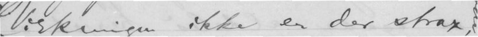Virkningen ikke er der strax,
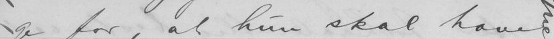ge for, at hun skal have
